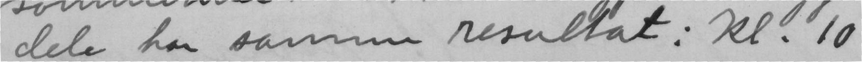dele har samme resultat: kl. 10
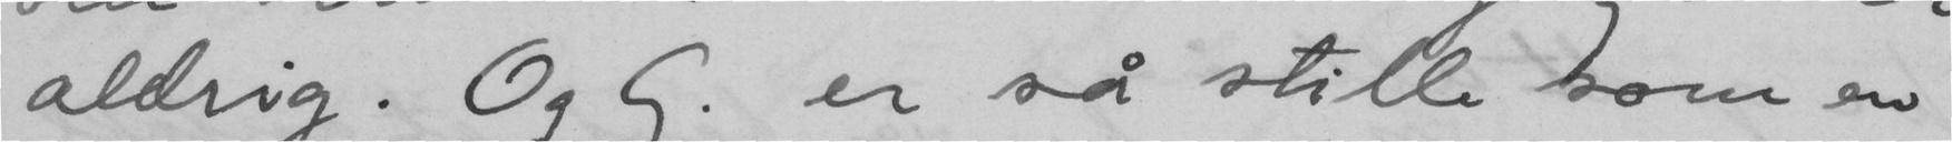aldrig. Og G. er så stille som en
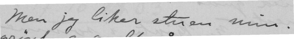Men jeg liker stuen min.
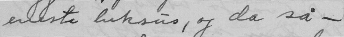eneste luksus, og da så -
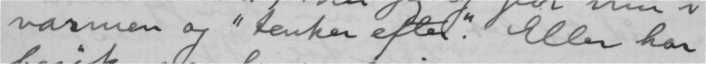varmen og "tenker efter". Eller har
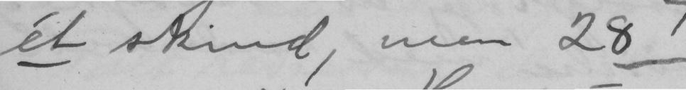ét skind, men 28!
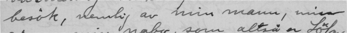besøk, nemlig av min mann, min
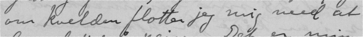om kvelden flotter jeg mig med at
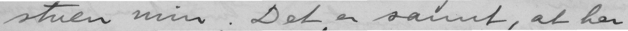stuen min. Det er sannt, at her
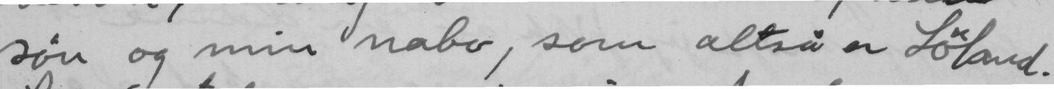søn og min nabo, som altså er Løland.
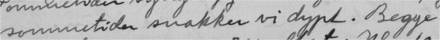sommetider snakker vi dypt. Begge
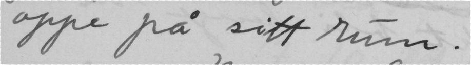oppe på sitt rum.
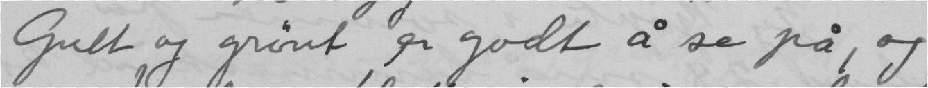Gult og grønt er godt å se på, og
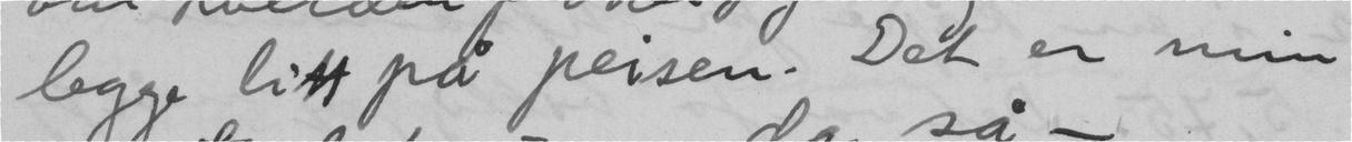legge litt på peisen. Det er min
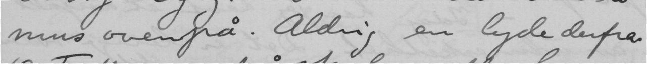mus ovenpå. Aldrig en lyde derfra.
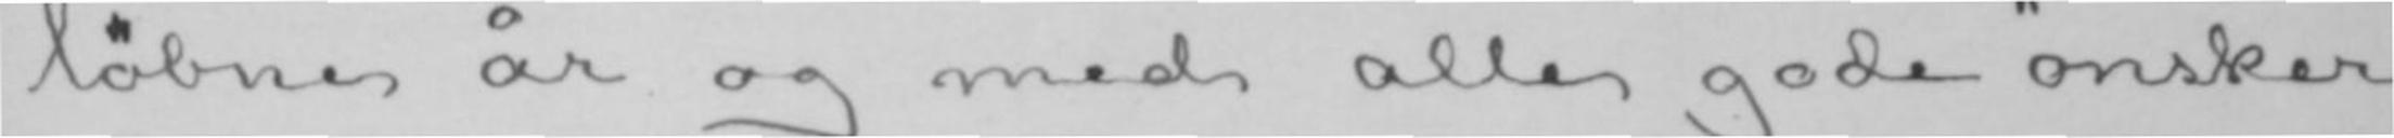løbne år og med alle gode ønsker
